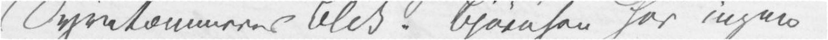Dyretæmmeres Blik. Bjørnson har ingen
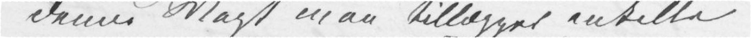denne Magt man tillægger enkelte
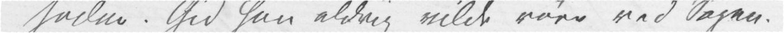sådan. Gid han aldrig vilde røre ved Sagen.
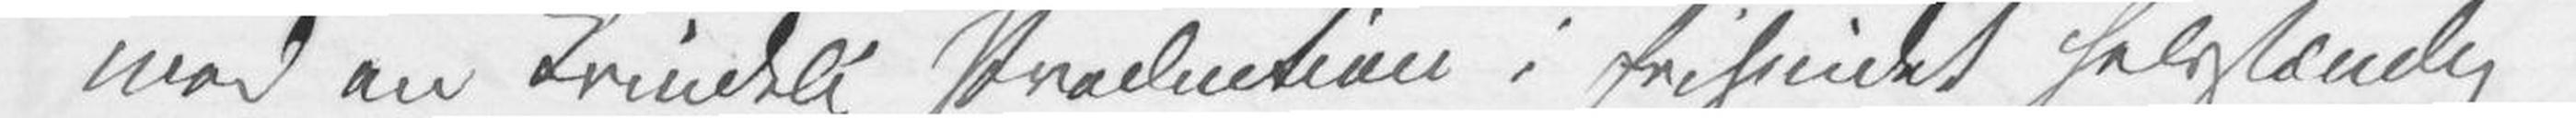mod en kvindelig Production i frisindet selvstændig
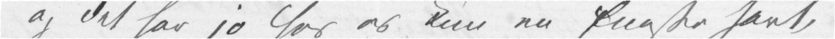og det har jo for os kun en Eneste havt,
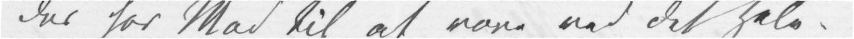der har Mod til at røre ved det Hele,
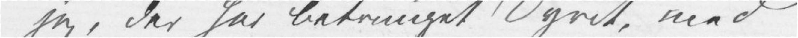jeg, der har betvunget Dyret, med
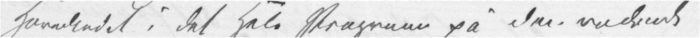Hovedlodet i det Hele Program på den rådende
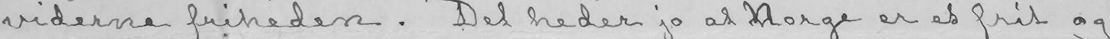viderne friheden. Det heder jo at nNorge er et frit og
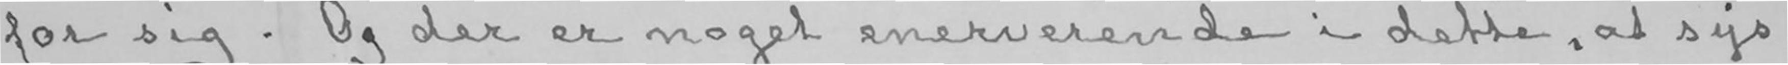for sig. Og der er noget enerverende i dette, at sys-
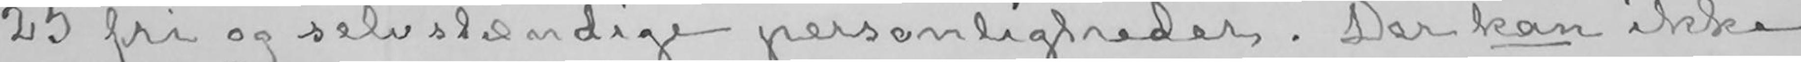25 fri og selvstændige personligheder. Der kan ikke
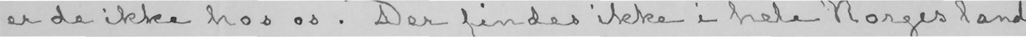er de ikke hos os. Der findes ikke i hele Norges land
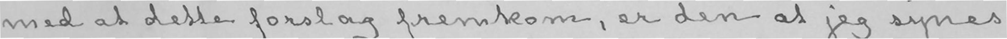med at dette forslag fremkom, er den at jeg synes
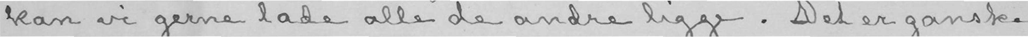kan vi gerne lade alle de andre ligge. Det er ganske
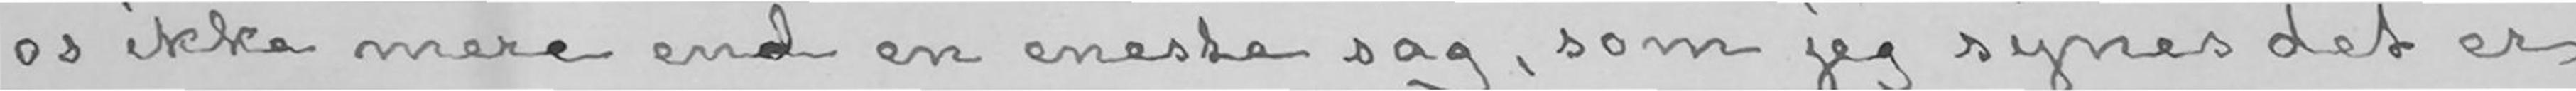os ikke mere end en eneste sag, som jeg synes det er
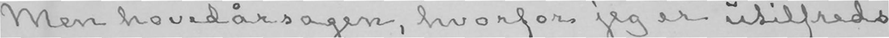Men hovedårsagen, hvorfor jeg er utilfreds
